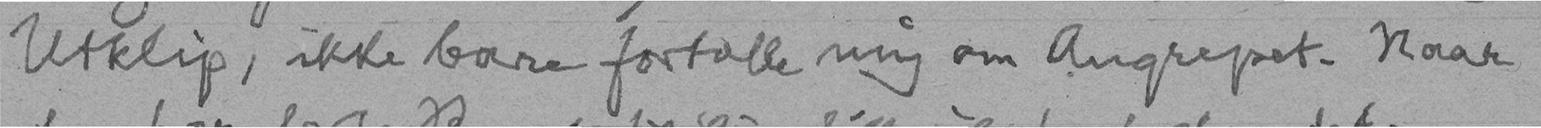Utklip, ikke bare fortælle mig om Angrepet. Naar
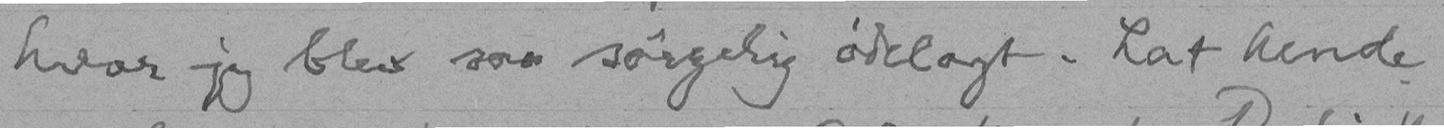hvor jeg blev saa sørgelig ødelagt. Lat hende
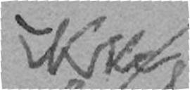ikke
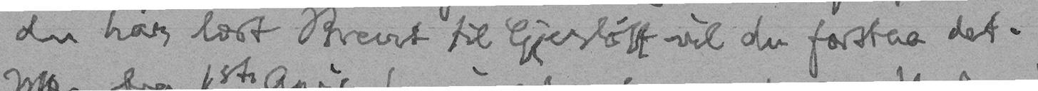du har læst Brevet til Gierløff vil du forstaa det.
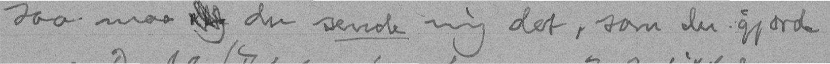saa maa  du sende mig det, som du gjorde
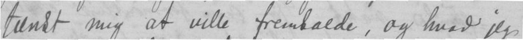mig at ville fremkalde, og hvad jeg
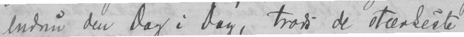endnu den Dig i dag, trods de stærkeste
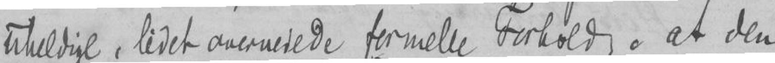uheldige, lidet over formelle Forhold at den
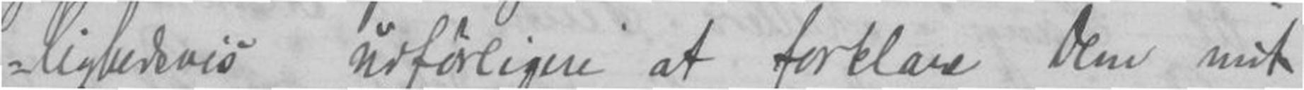lighedsvis udførligne at forklare dem mit
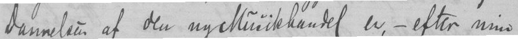Dannelsen af den nye Musikhandel er,- efter min
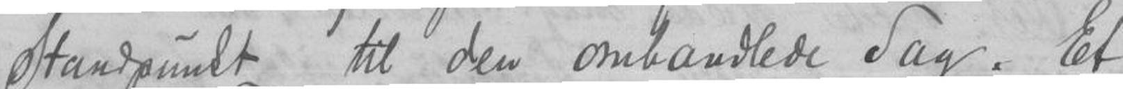Standpunkt til den omhandlede Sag. Et
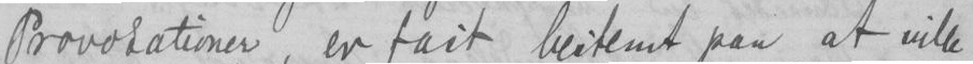Provokationer, er fast bestemt paa at ville
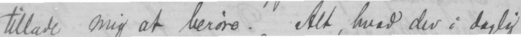tillade mig at berøre. Alt, hvad der i daglig
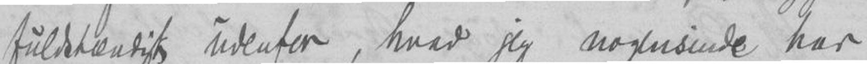juldstændigt udenfor, hvad jeg nogensinde har
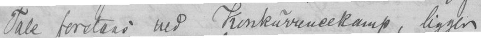Tale forstaas med kunkurrencekamp, ligger
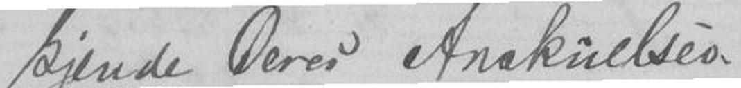kjende Deres Anskuelser.
